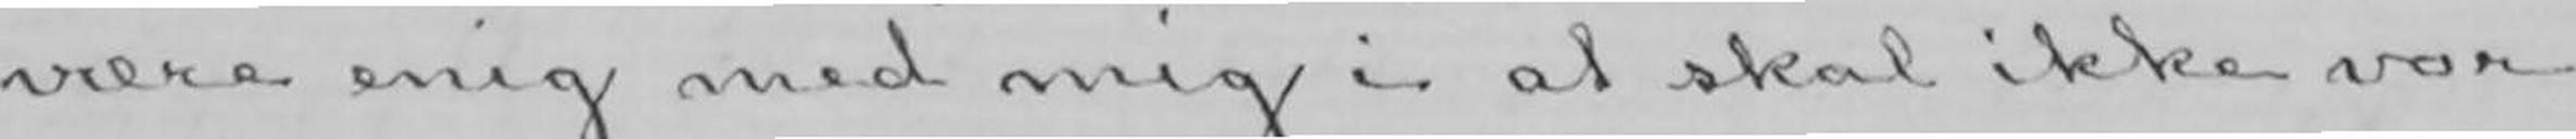være enig med mig i at skal ikke vor
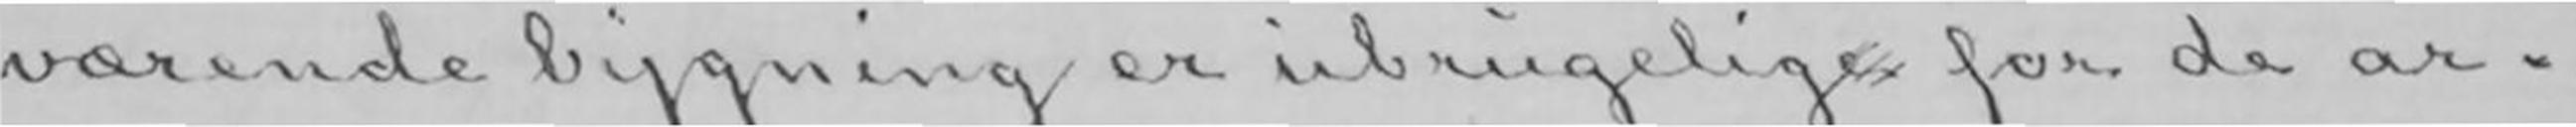værende bygning er ubrugelige for de ar-
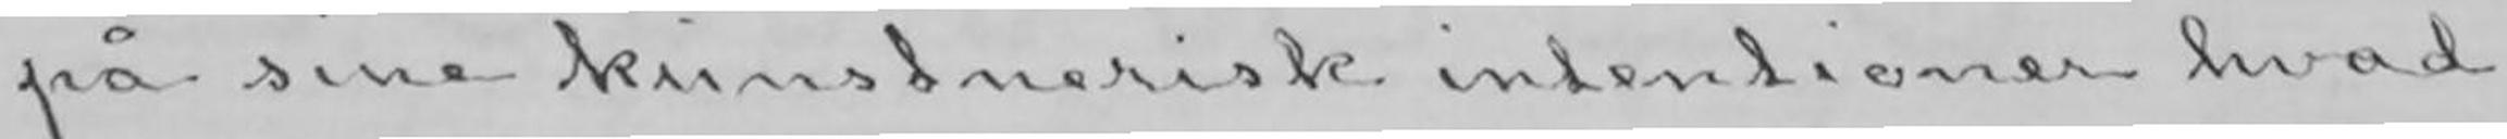på sine kunstnerisk intentioner hvad
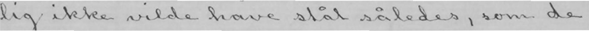lig ikke vilde have ståt således, som de
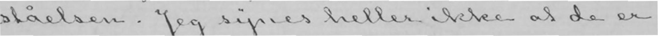ståelsen. Jeg synes heller ikke at de er
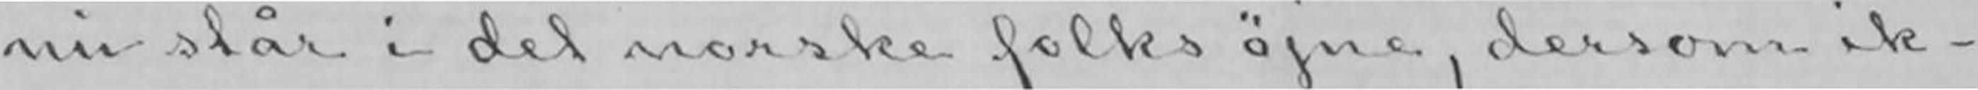nu står i det norske folks øjne, dersom ik-
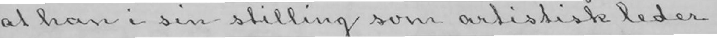at han i sin stilling som artistisk leder
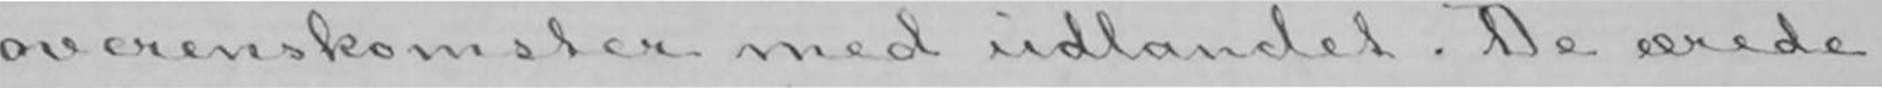overenskomster med udlandet. De ærede
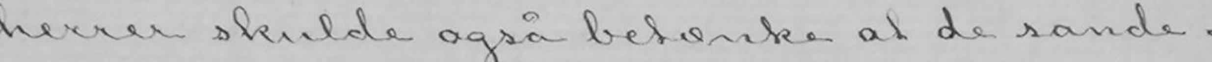herrer skulde også betænke at de sande-
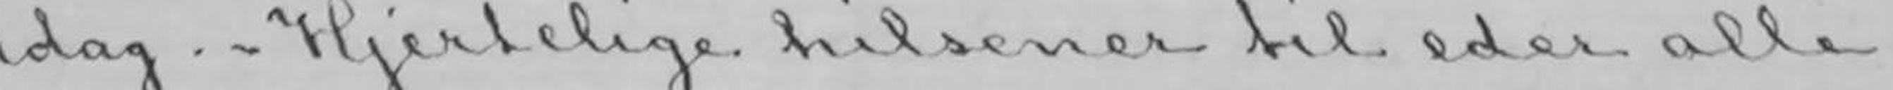idag.- Hjertelige hilsener til eder alle.
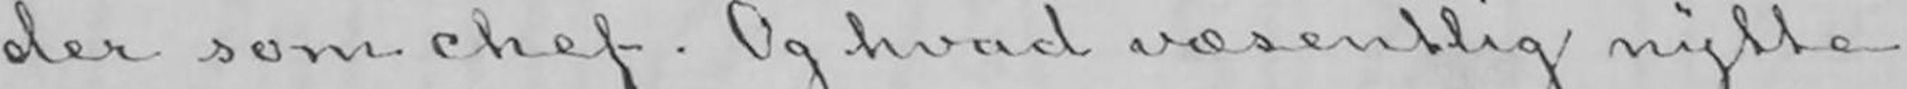der som chef. Og hvad væsentlig nytte
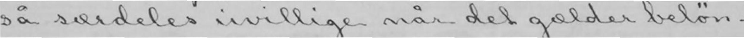så særdeles uvillige når det gælder beløn-
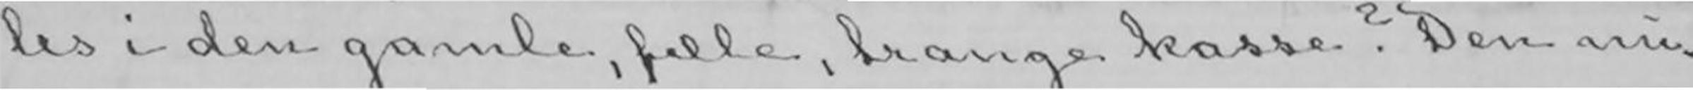les i den gamle, fæle, trange kasse? Den nu-
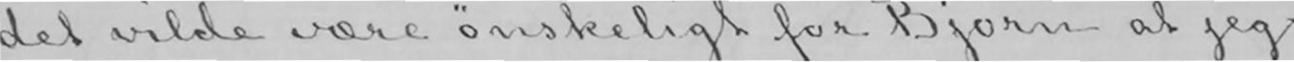det vilde være ønskeligt for Bjørn at jeg
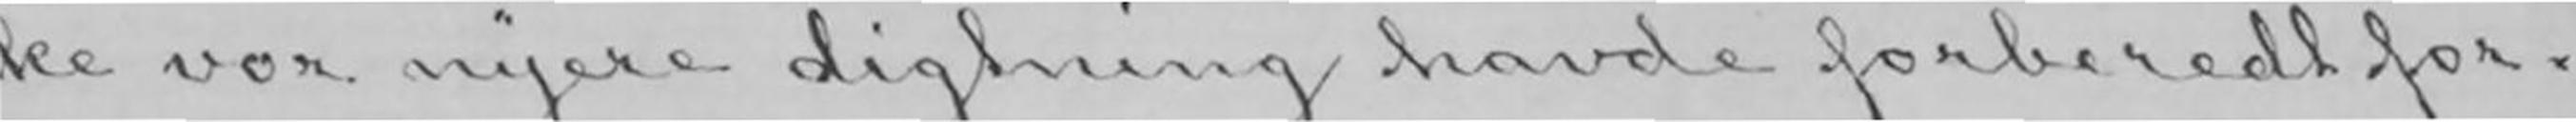ke vor nyere digtning havde forberedt for-
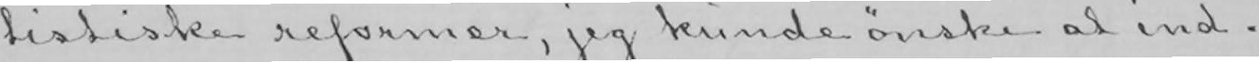tistiske reformer, jeg kunde ønske at ind-
